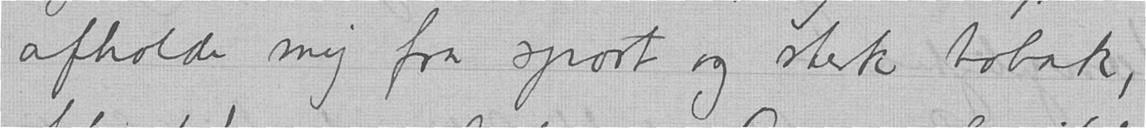afholde mig fra sport og sterk tobak,
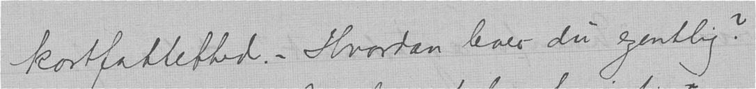kortfattethed. — Hvordan lever du egentlig?
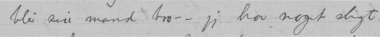blir sin mand tro — — jeg har noget sligt
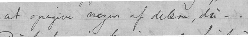ikke at opgive nogen af delene, du —.
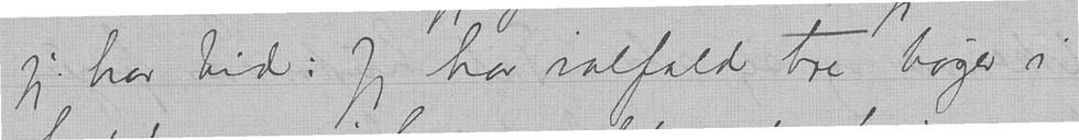jeg har tid: Jeg har ialfald tre bøger i
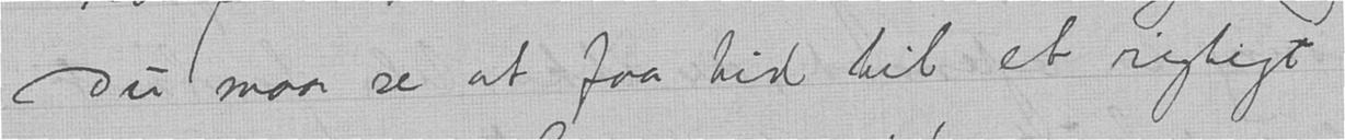Du maa se at faa tid til et rigtigt
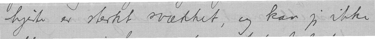hjerte er sterkt svækket, og kan jeg ikke
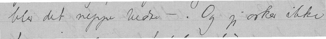blir det neppe bedre —. Og jeg orker
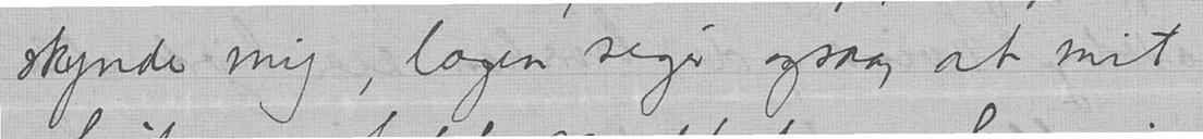skynde mig, lægen siger ogsaa at mit
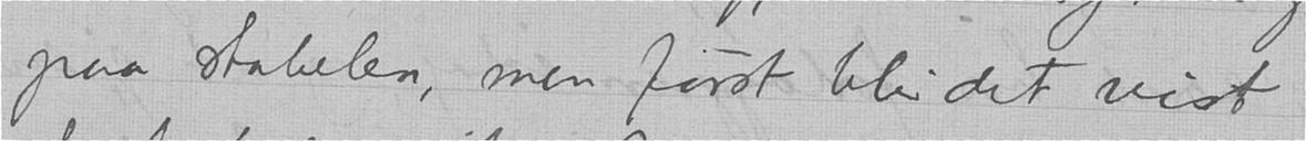paa stabelen, men først blir det vist
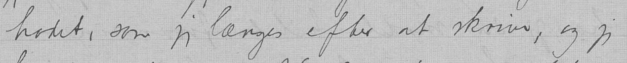hodet, som jeg længes efter at skrive, og jeg
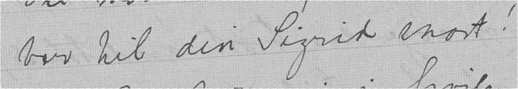brev til din Sigrid snart!
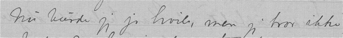Nu burde jeg jo hvile, men jeg tror ikke,
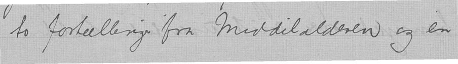to fortællinger fra Middelalderen og en
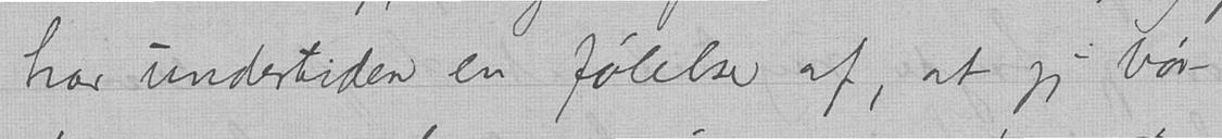har undertiden en følelse af, at jeg bør
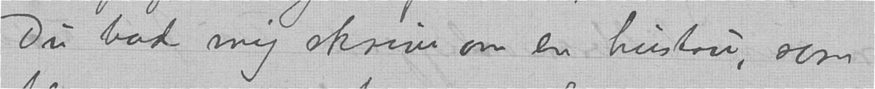Du bad mig skrive om en hustru, som
